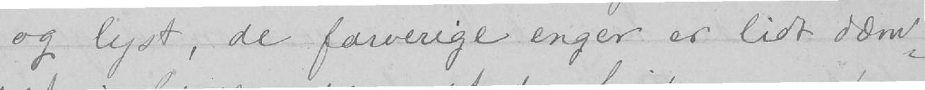og lyst, de farverige enger er lidt dæm-
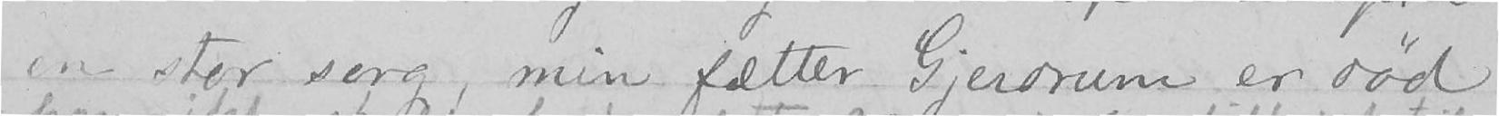en stor sorg, min fætter Gjerdrum er død
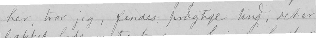her, tror jeg, findes prægtige ting, det er
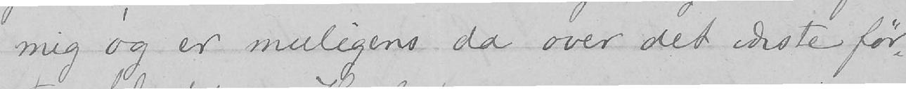mig og er muligens da over det værste før-
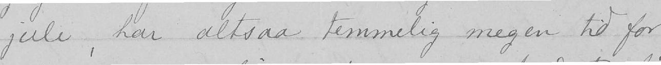juli, har altsaa temmelig megen tid for
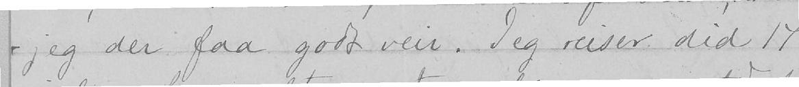jeg der faa godt veir. Jeg reiser did 17
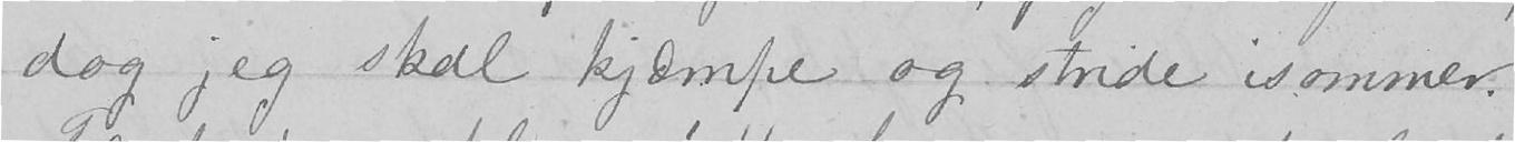dog jeg skal kjæmpe og stride isommer.
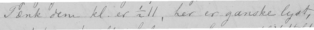Tænk dem kl. er ½11, her er ganske lyst,
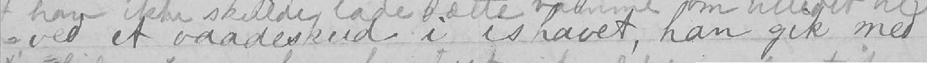ved et vaadeskud i ishavet, han gik med
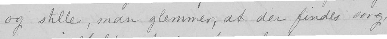og stille, men glemmer, at der findes sorg,
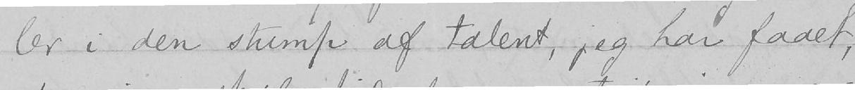ler i den stump af talent, jeg har faaet,
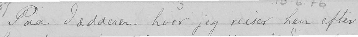Paa Jædderen hvor jeg reiser hen efter
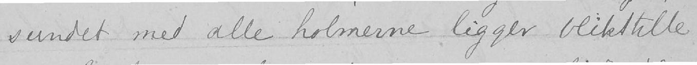sundet med alle holmerne ligger blikstille
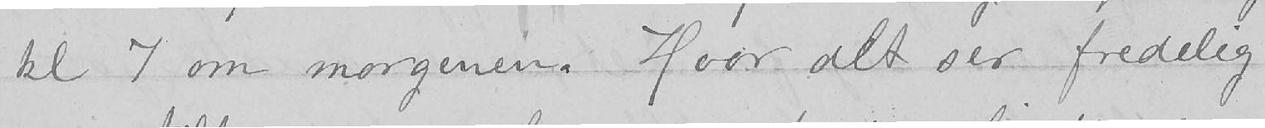kl 7 om morgenen. Hvor alt ser fredelig
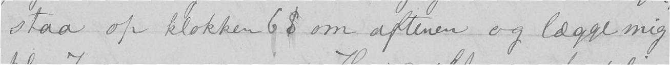staa op klokken 6 S om aftenen og lægge mig
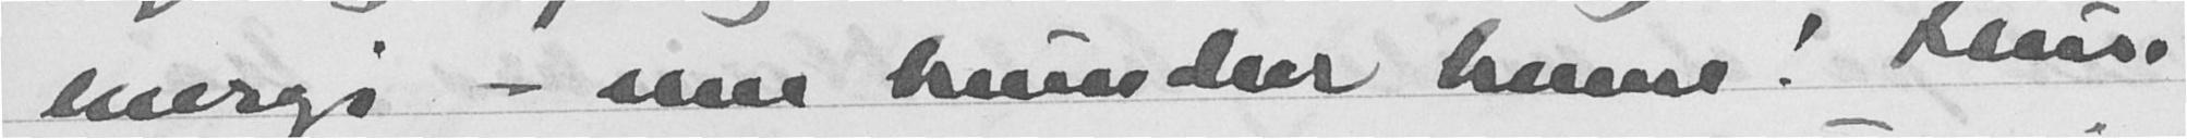energi - men beundrer henne! Hun
